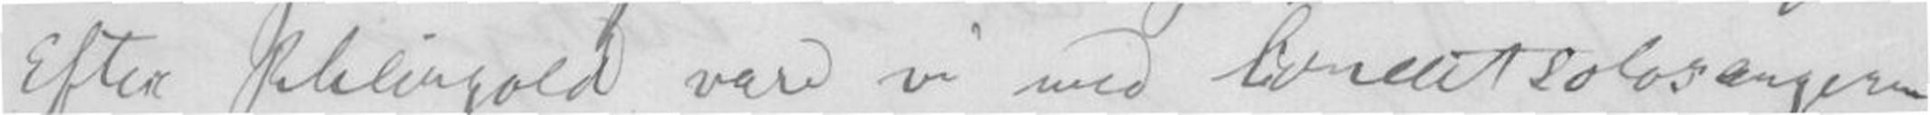Efter Rheingold var vi med Concertsolosangerne
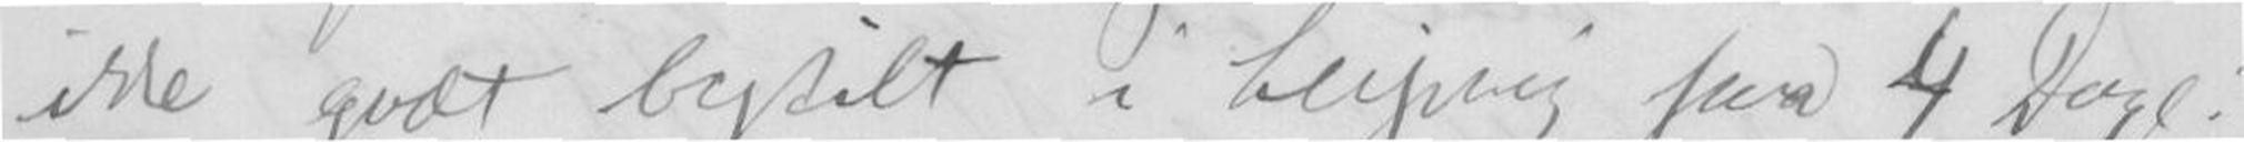ikke godt bestilt i Leipzig for 4 Dage.
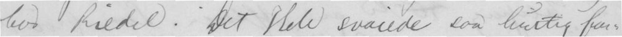hos Riedel. Det Hele svaiede saa hurtig for
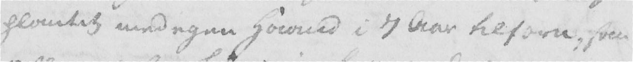plantet med egen Haand i 7 Aar tilforn, som
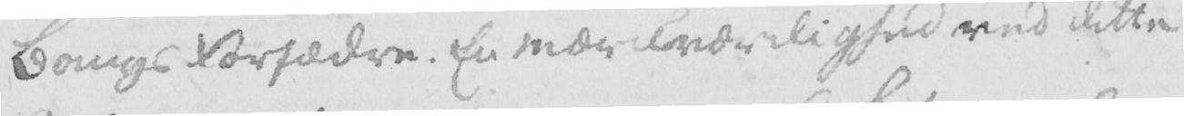Bangs Forfædre. En Mærkverdighed ved dette
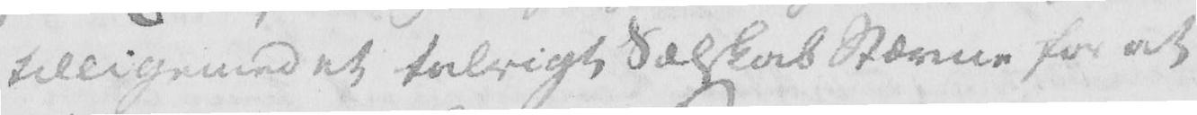tilligemed et talrigt Sælskab Stævne for at
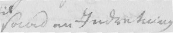saad en Indretning
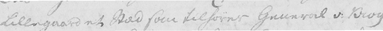Lillegaard et Stæd som tilhører General v. Krog
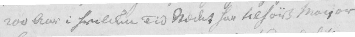200 Aar i hvilken Tid stædet har tilhørt Major
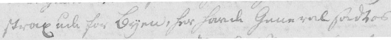strax ude for Byen, her havde General sadt os
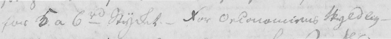for 5 a 6 rd Stycket. - For Oeconomiens skyld lig-
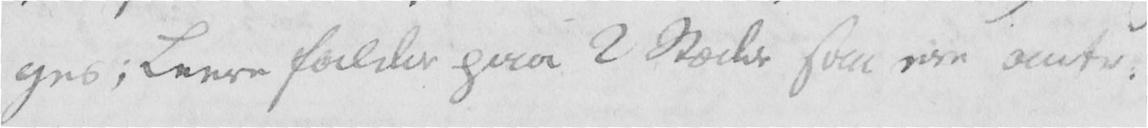ges; Leere falder paa 2 Stæder som ere omtr.
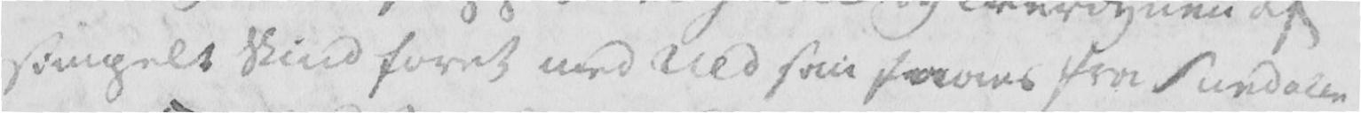simpelt Skind foret med Uld som faaes fra Sundalen
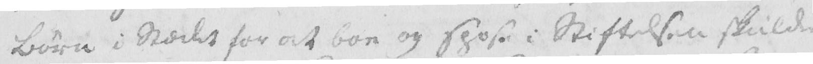Børn i Stædet for at boe og spise i Stiftelsen skulde
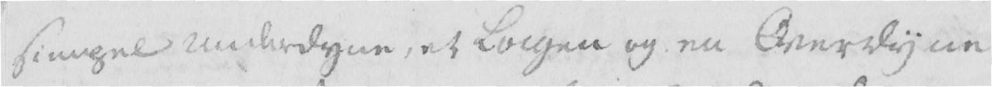simpel Underdyne, et Lagen og en Overdyne
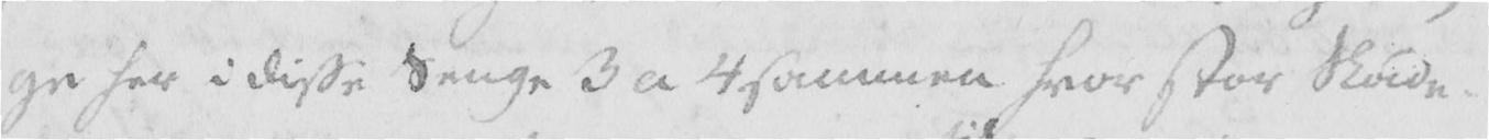ge her i disse Senge 3 a 4 sammen hvor stor Skade-
